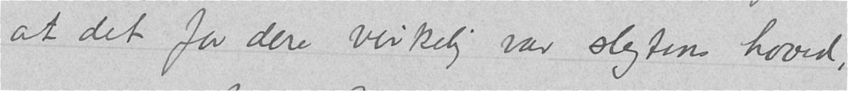at det for dere virkelig var slegtens hoved,
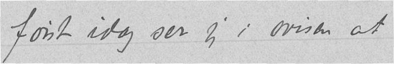først idag ser jeg i avisen at
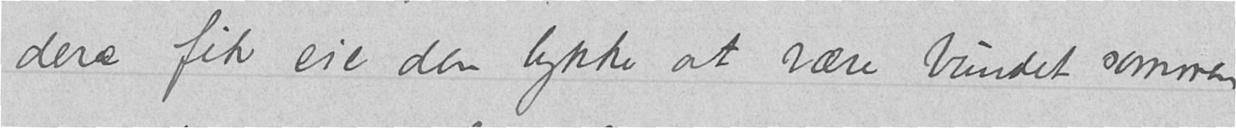dere fik eie den lykke at være bundet sammen
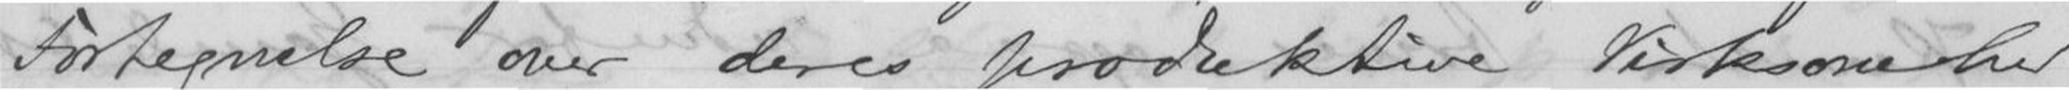Fortegnelse over deres produktive Virksomhed
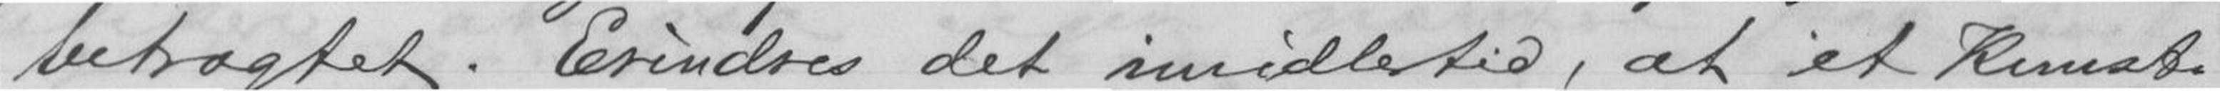betragtet. Erindres det imidlertid, at et Kunst-
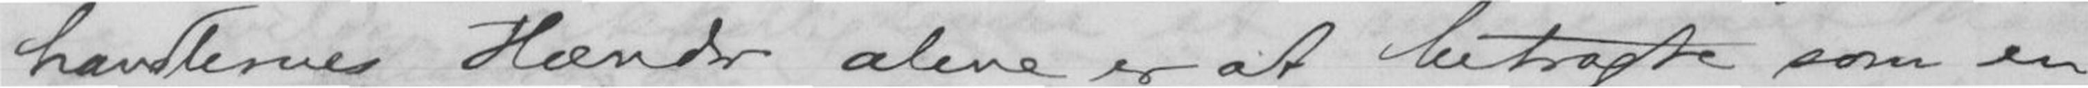handlernes Hænder alene er at betragte som en
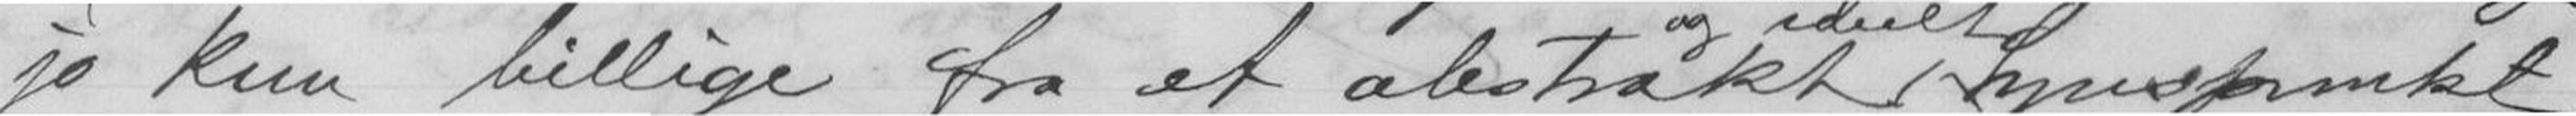jo kun billige fra et abstrakt Synspunkt
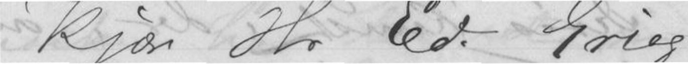Kjære Hr Ed. Grieg,
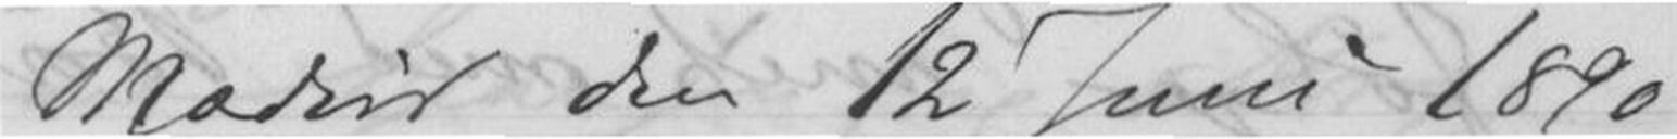Madrid den 12 Juni 1890
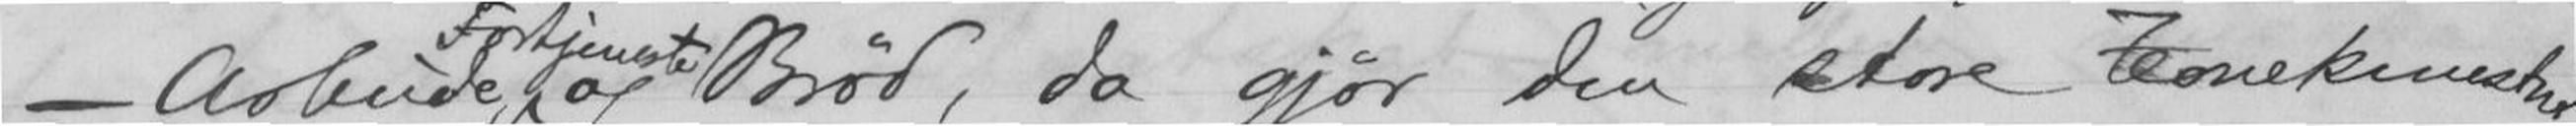- Arbeide, og Brød, da gjør den store Tonekunstner
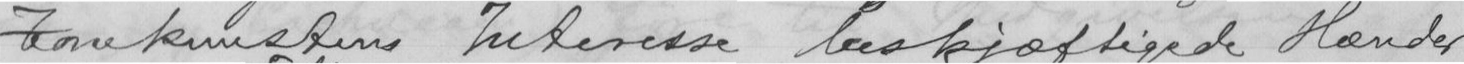Tonekunstens Interesse beskjæftigede Hænder
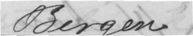Bergen
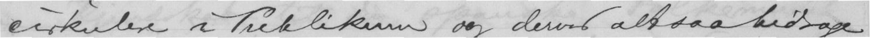cirkulere i Publikum og derved altsaa bidrage
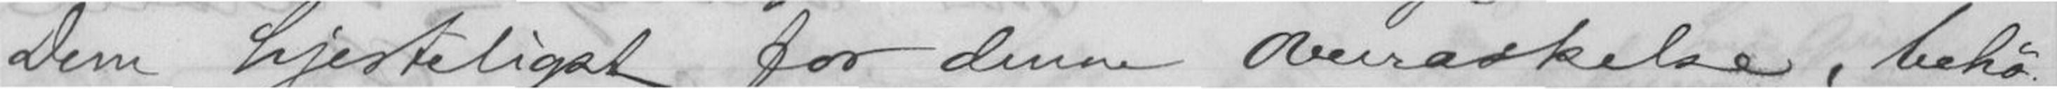Dem hjerteligst for denne Overraskelse, behø-
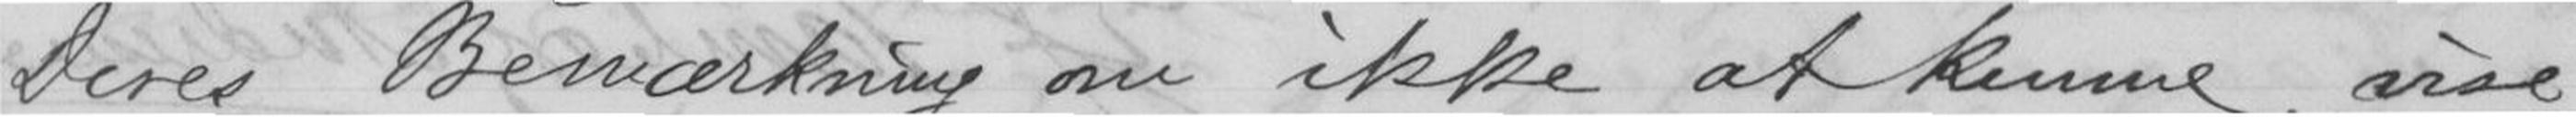Deres Bemærkning om ikke at kunne vise
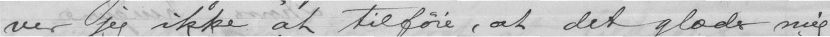ver jeg ikke at tilføie, at det glæder mig
